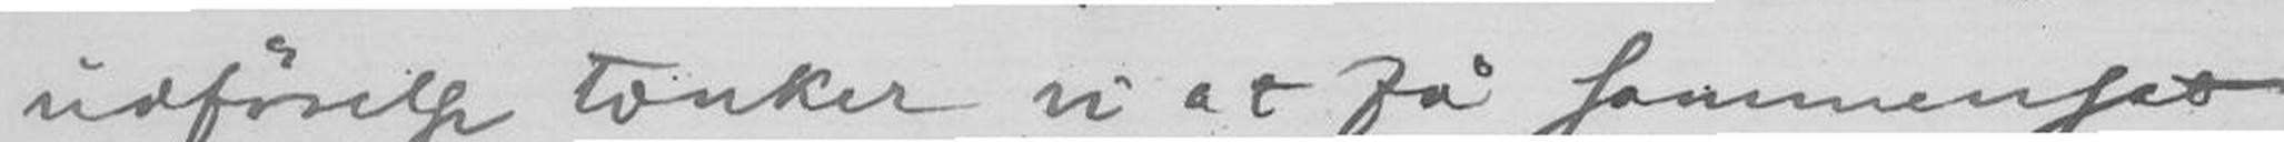udførelse tænker vi at få sammensat
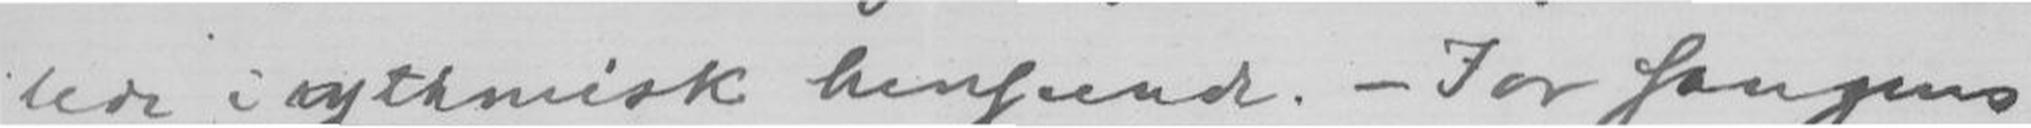lede i rythmisk henseende. - For sangens
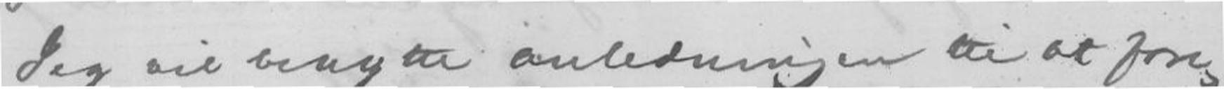Jeg vil benytte anledningen til at fore-
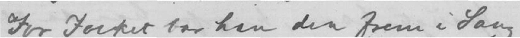For Folket bar han den frem i Sang
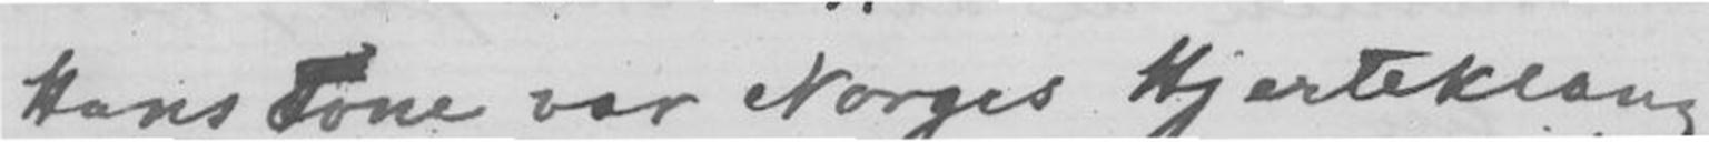Hans Tone var Norges Hjerteklang
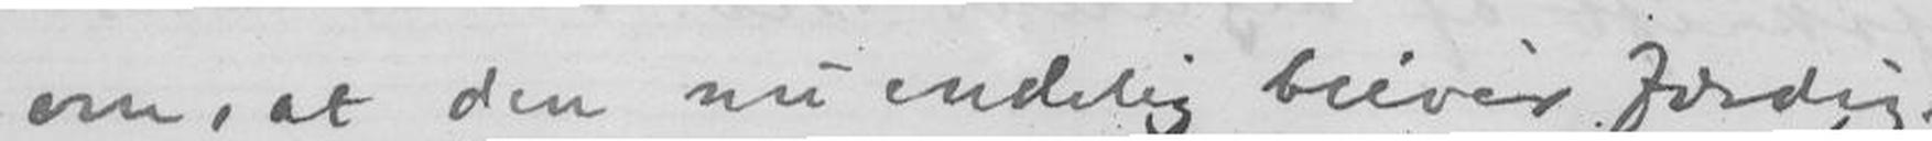om, at den nu endelig bliver færdig i
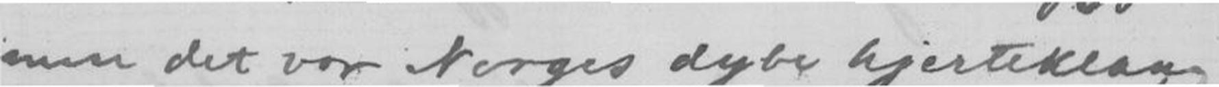men det var Norges dybe hjerteklang,
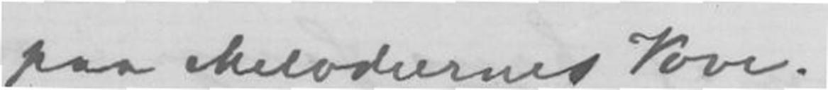paa Melodiernes Vove.
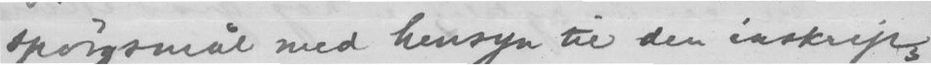spørgsmål med hensyn til den inskrip-
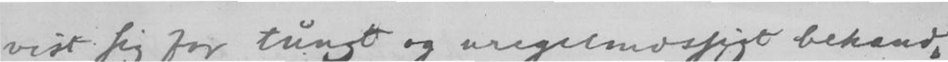vist sig for tungt og uregelmæssigt behand-
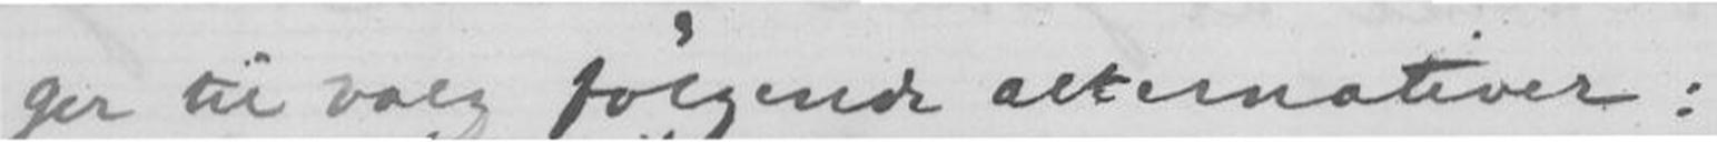ger til valg følende alternativer:
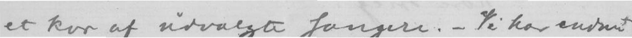et kor af udvalgte sangere. - Vi har endnu
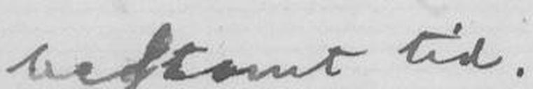bestæmt tid.
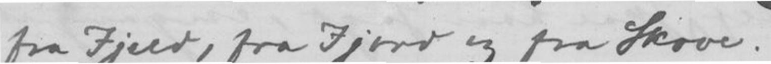fra Fjeld, fra Fjord og fra Skove.
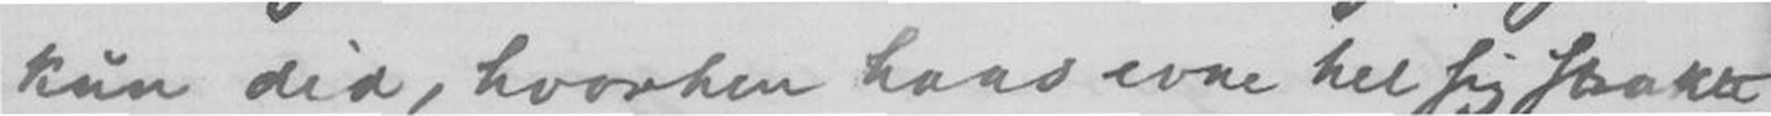kun did, hvorhen hans evne hel sig strakte,
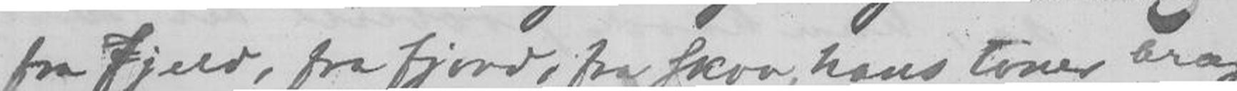fra Fjeld, fra fjord, fra skov, hans toner bragte.
